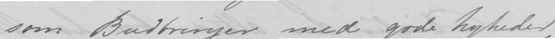som Budbringer med gode nyheder,
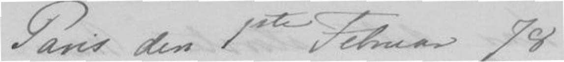Paris den 1ste Februar 78
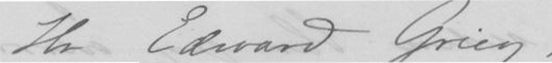Hr Edvard Grieg.
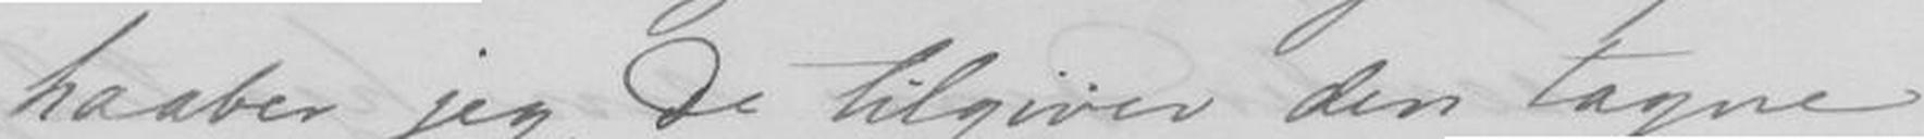haaber jeg De tilgiver den tagne
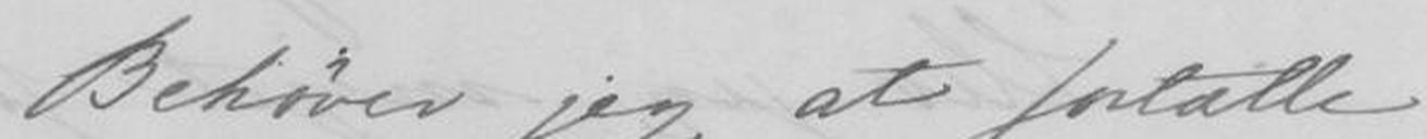Behøver jeg at fortælle
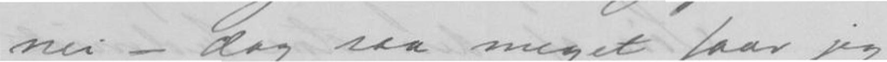nei - dog saa meget faar jeg
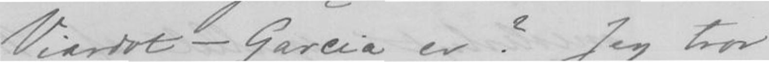Viardot-Garcia er? Jeg tror
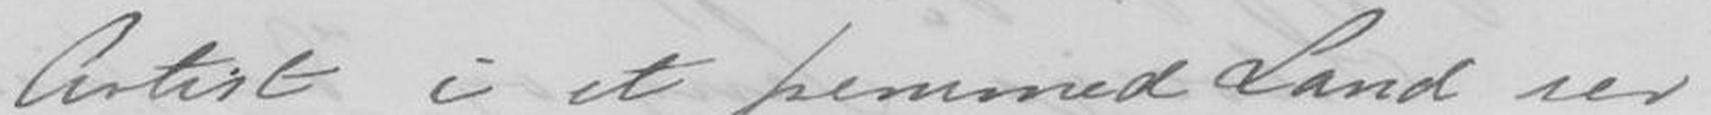Artist i et fremmed Land ser
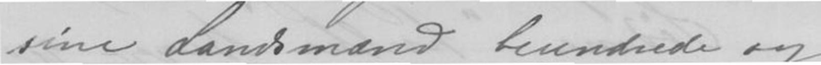sine Landsmænd beundrede og
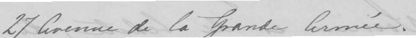21 Avenue de la Grande Armée
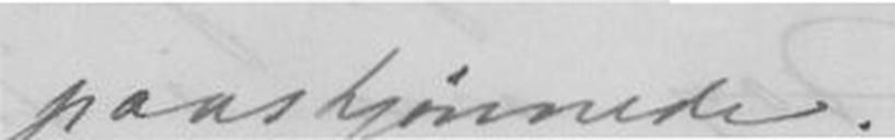paaskjønnede.
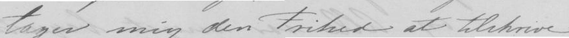tager mig den Frihed at tilskrive
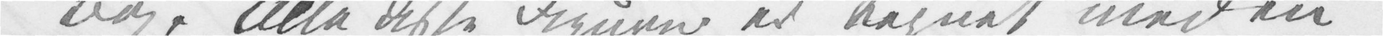Bog. Alle disse Figurer er tegnet med en
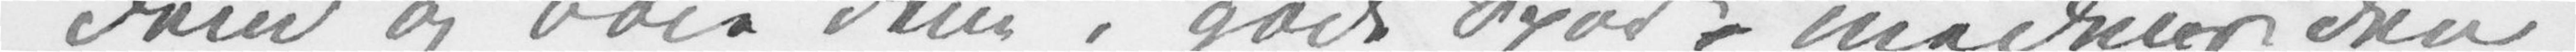dem og bøie dem i gode Spor, medens den
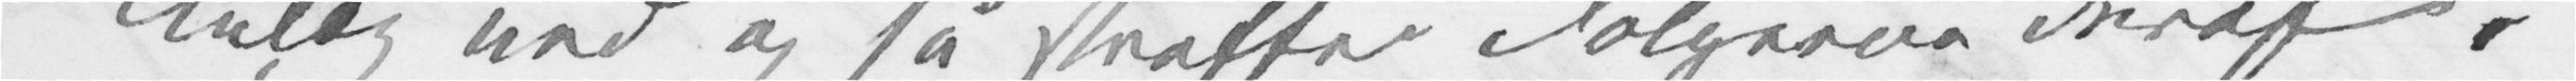Anlæg ned og så straffe Følgerne deraf.
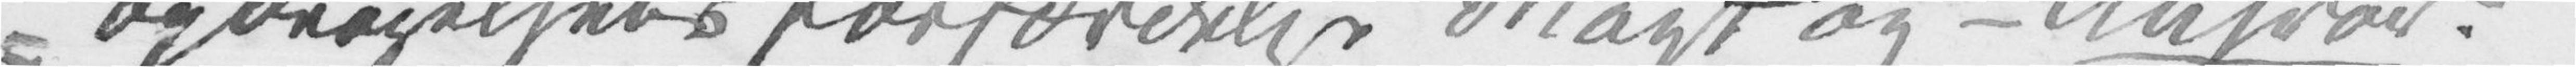opdragelsens forfærdelige Magt og – Ansvar,
